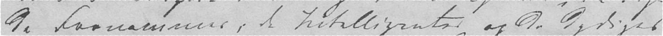de Fornemmes, de Intelligentes og de Dydiges
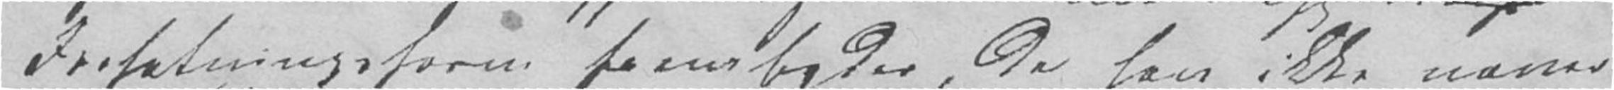Forfatningsform frembyder, da han ikke X
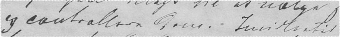og controllere dem. Imidlertid
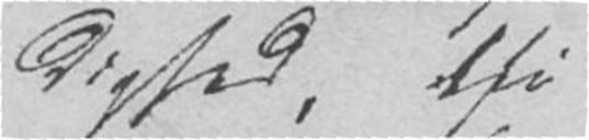dighed, thi
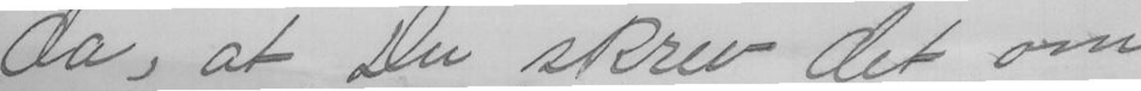da, at Du skrev det om
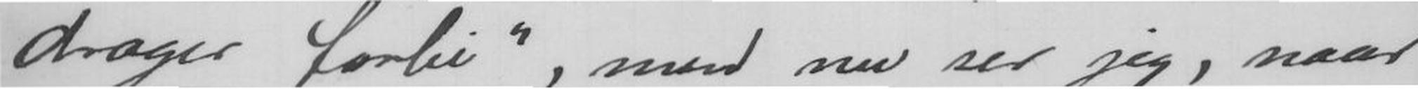drager forbi", men nu ser jeg, naar
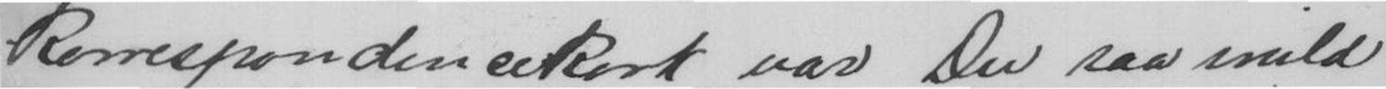korrespondencekort var Du saa snild
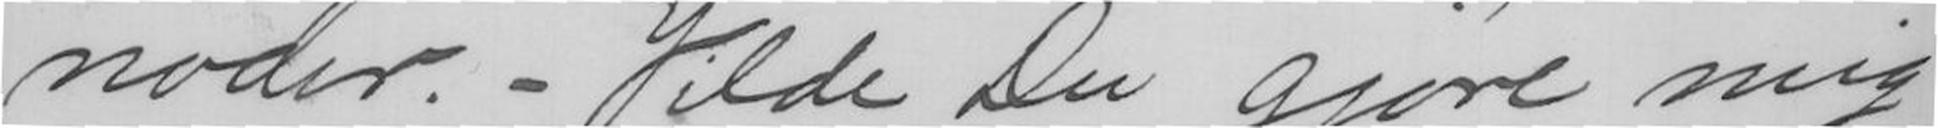noder. - Vilde Du gjøre mig
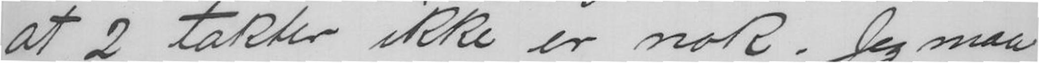at 2 takter ikke er nok. Jeg maa
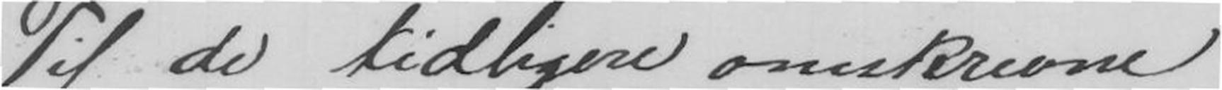Til de tidligere omskrevne
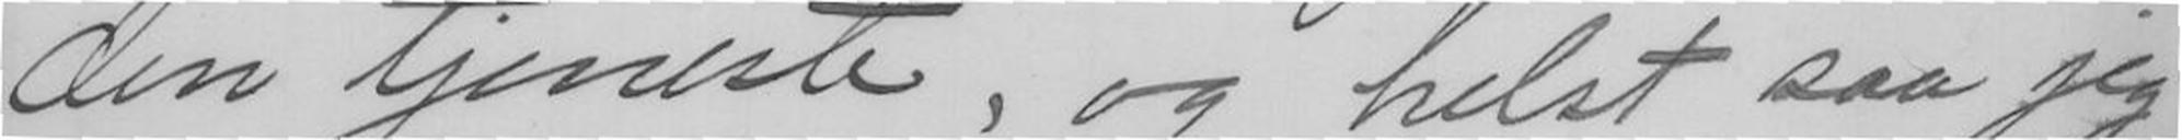den tjeneste, og helst saa jeg
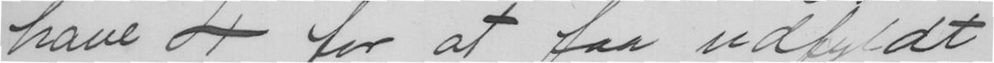have 4 for at faa udfyldt
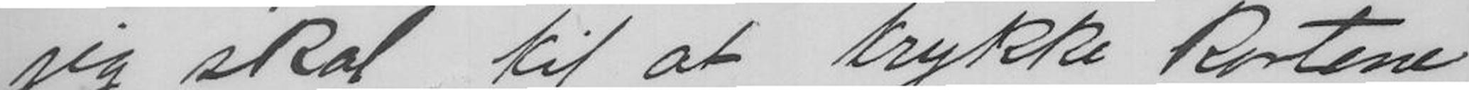jeg skal til at trykke kortene
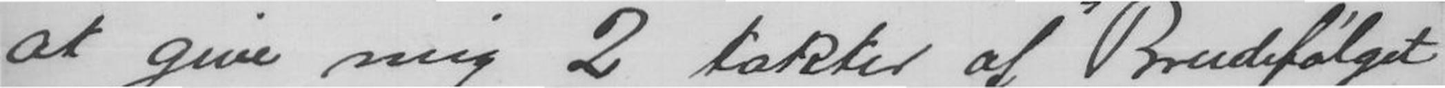at give mig 2 takter af "Brudefølget
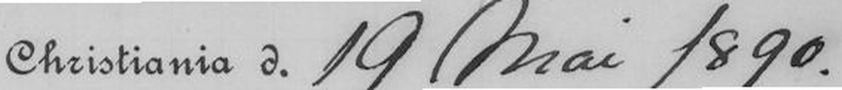Christiania d. 19 Mai 1890.
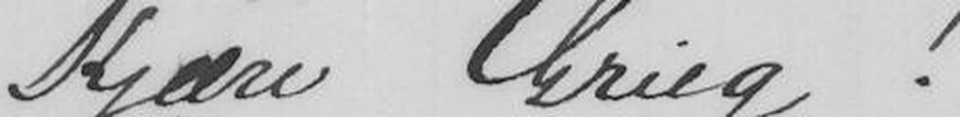Kjære Grieg!
